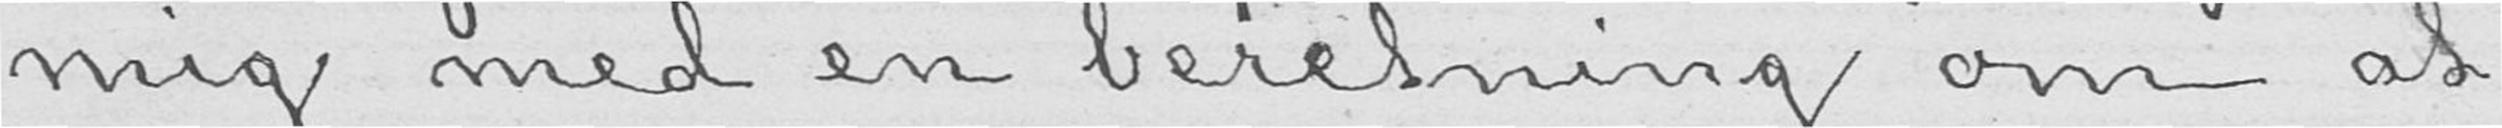mig med en beretning om at
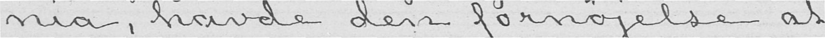nia, havde den fornøjelse at
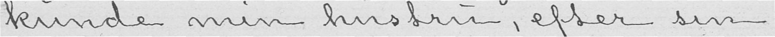kunde min hustru, efter sin
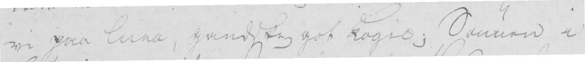vi paa lena, gandske got Logie; Sønnen i
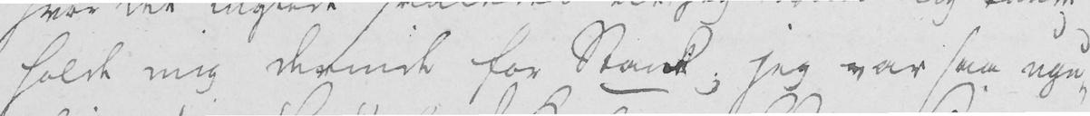holde mig derinde for Stank; jeg var saa ugu-
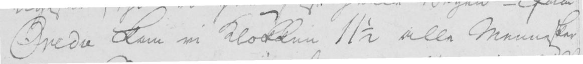Credu kom vi Klokken 11 1/2 alle Mennesker
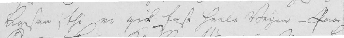ligesaa, thi vi gik fast heele Veyen - Paa
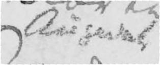Lne lndan
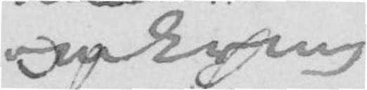omkring
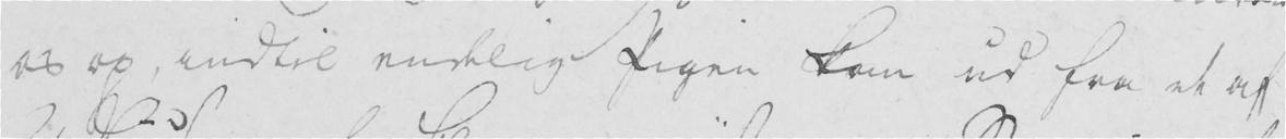os op, indtil endelig Pigen kom ud fra et af
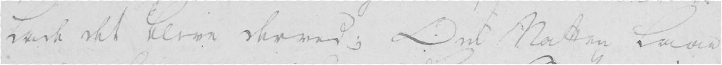lade det blive derved; Om Natten laae
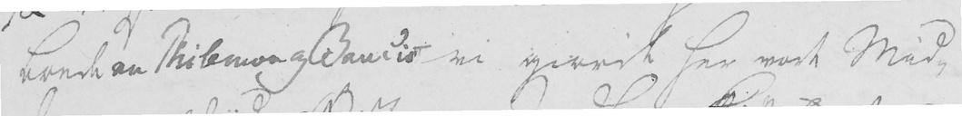Bonden Philemon og Baucis vi giorde her vort Mid-
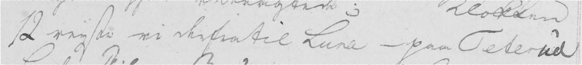12 reyste vi derfra til Lena paa Teterud
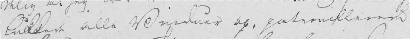lukkede alle Vinduer og patrouillerede
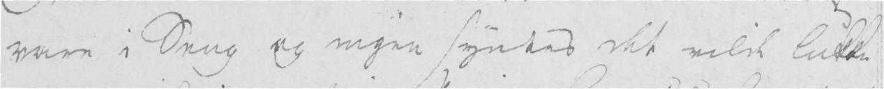vare i Seng og ingen syntes det vilde lukke
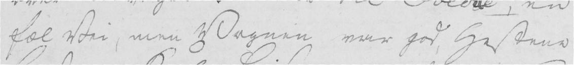fæl Vei, men Vognen var god, Hestene
